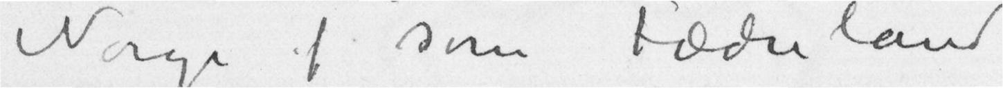Norge som Fædreland
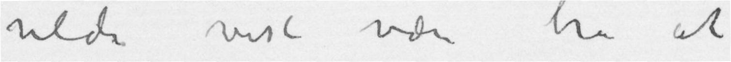vilde vist være bra at
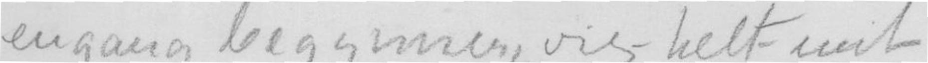engang begynner, vies helt mit
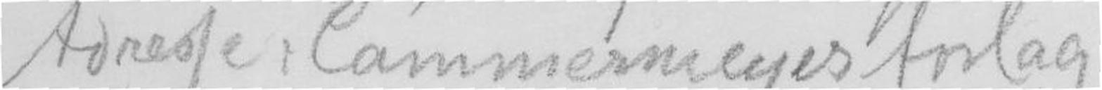Adresse; Cammermeyer forlag.
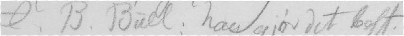J. B. Bull; han gjør det best.
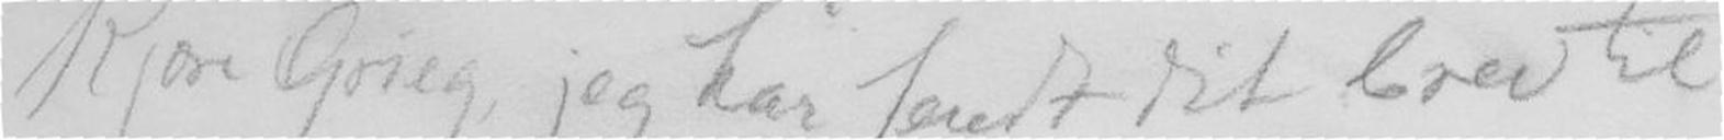Kjære Grieg jeg har sendt dit brev til
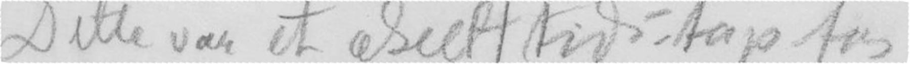Dette var et ækelt tids-tap for
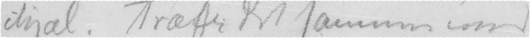ihjæl. Træffer det sammen med
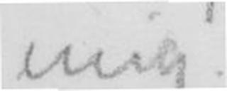mig.
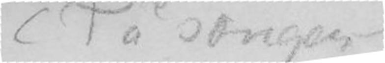(På sængen).
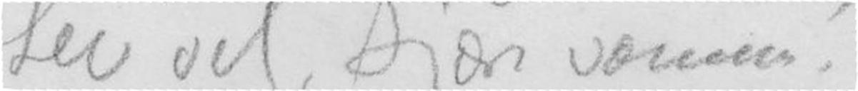Lev vel, kjære vænner!
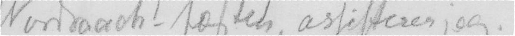Nordraach-fæsten, assisterer jeg.
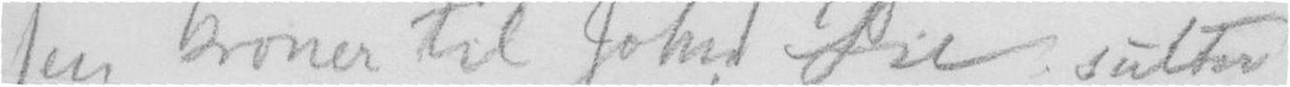sen kroner til John Lie; sulter
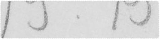B. B.
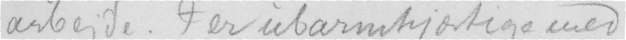arbejde. I er ubarmhjærtige med
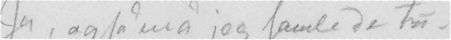Ja, og så må jeg samle de tu-
